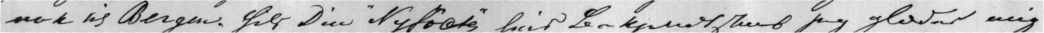nok til Bergen. Hils Din "Nyfødte" hvis Bekjendtskab jeg glæder mig
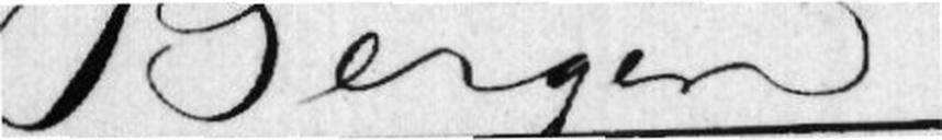Bergen
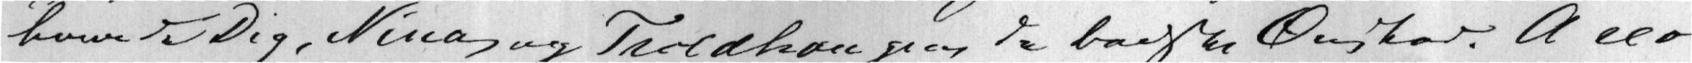baade Dig, Nina og Troldhaugen de Bedste Ønsker. Acco
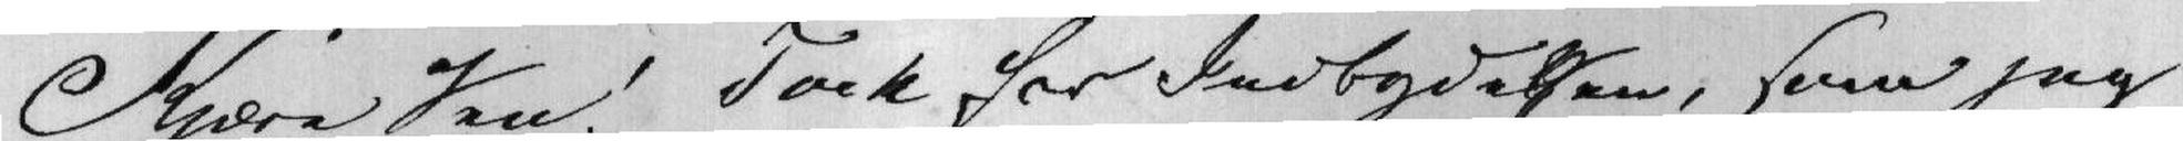Kjære Ven! Tack for Indbydelsen, som jeg
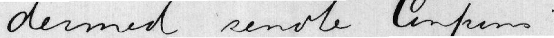dermed sendte Copien.
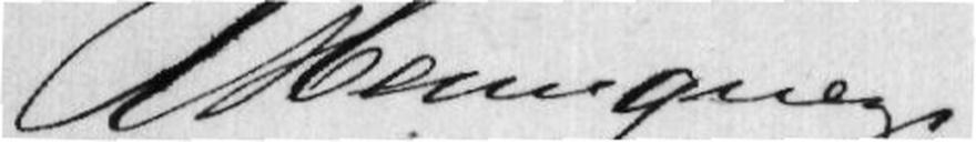A Henriques
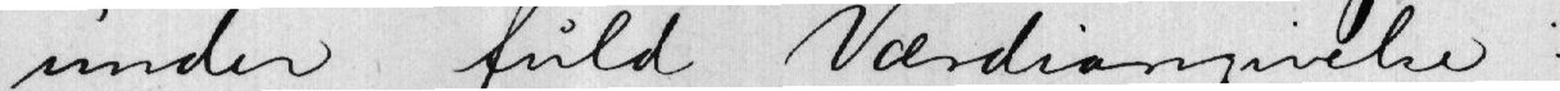under fuld Værdiangivelse:
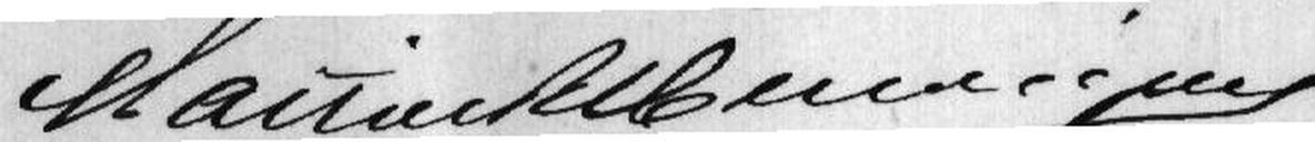Martin R Henriques
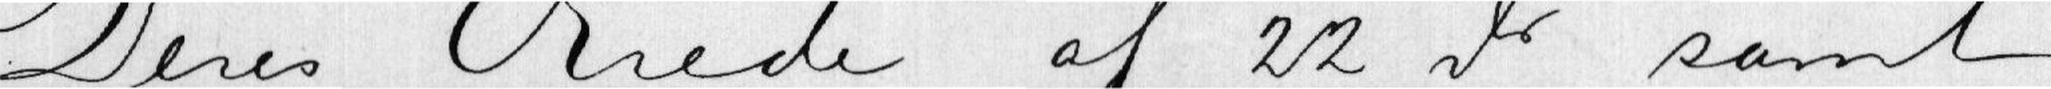Deres Erede af 22 do samt
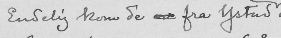Endelig kom de ov fra Ystad.
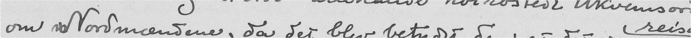om Nordmændene, da det blev betydet dem at det var en
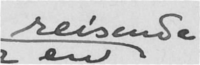reisende
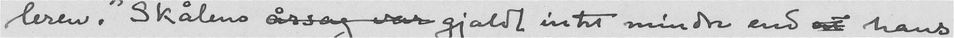leren." Skålens årsag var gjaldt intet mindre end at hans
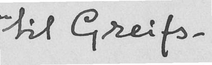til Greifs-
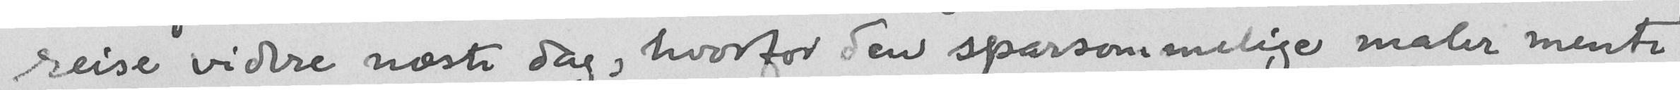reise videre næste dag, hvorfor den sparsommelige maler mente
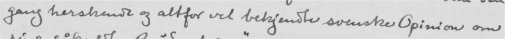gang herskende og altfor vel bekjendte svenske Opinion om
